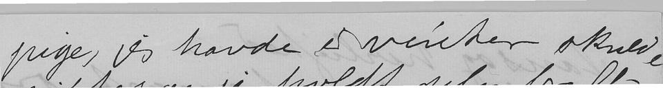pige, jeg havde i vinter skulde
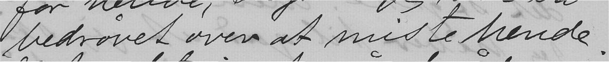bedrøvet over at miste hende.
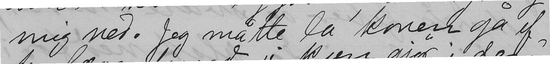mig ned. Jeg måtte la' konen gå ef-
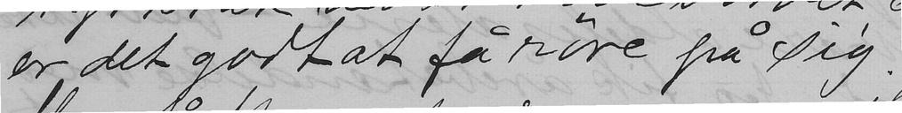er det godt at få røre på sig.
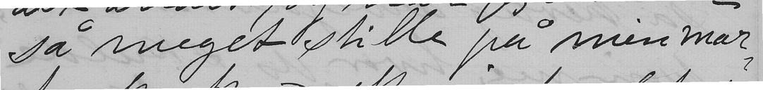så meget stille på min mar-
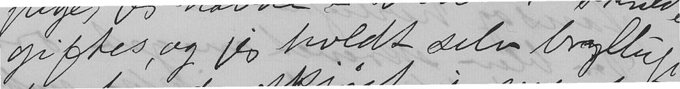giftes, og jeg holdt selv bryllup
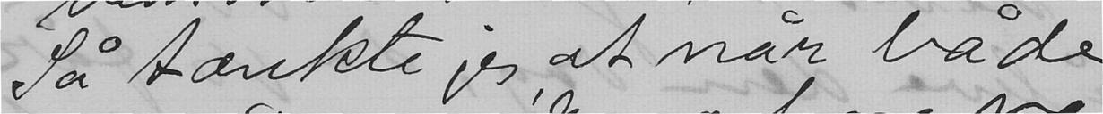Så tænkte jeg at når både
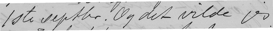1ste septbr. Og det vilde jeg
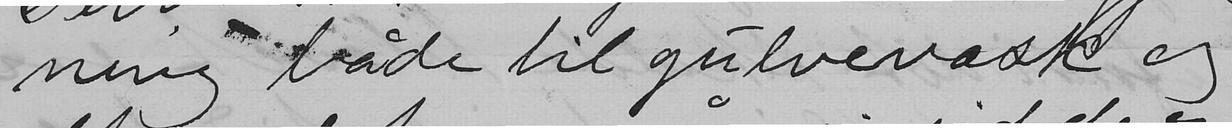ning både til gulvevask og
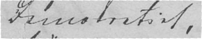Demokratiet,
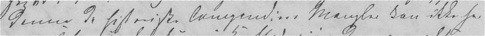denne de historiske Compendiers Mangler kan ikke her
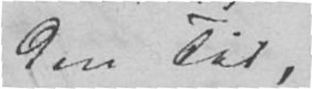den Tid,
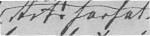Retsforfat-
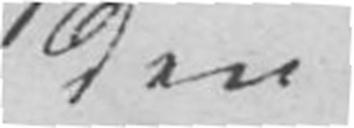den
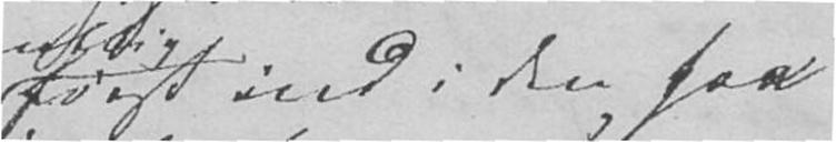først ind i den paa
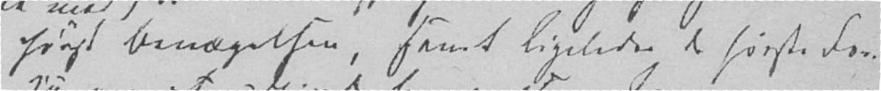først Bevægelsen, samt ligeledes de første For-
